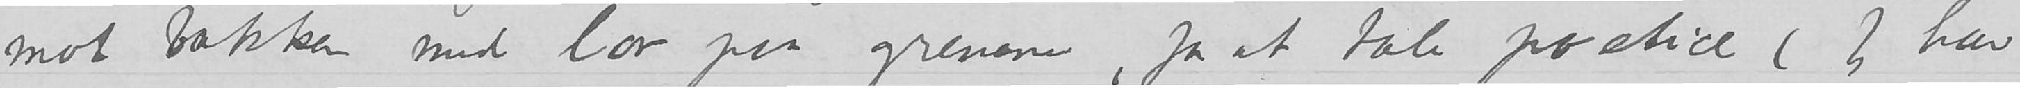mot bakken med lav paa grenerne, for at tale poeticc (jeg har
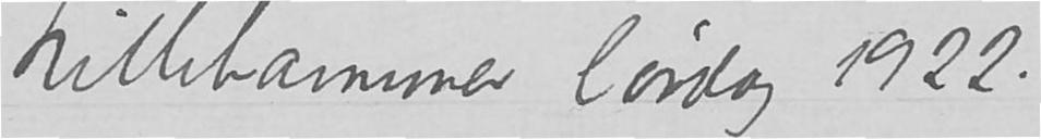Lillehammer lørdag 1922.
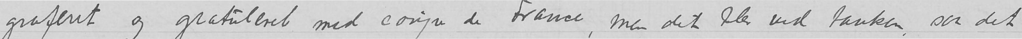graferet og gratuleret med coupe de France, men det blev ved tanken, saa det
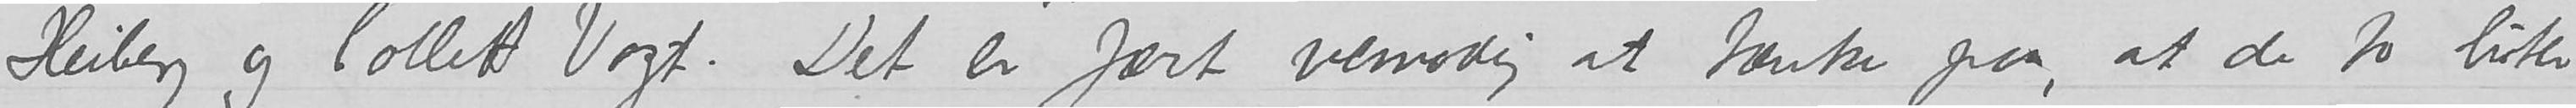Heiberg og Collett Vogt. Det er fært vemodig at tænke paa, at de to luter
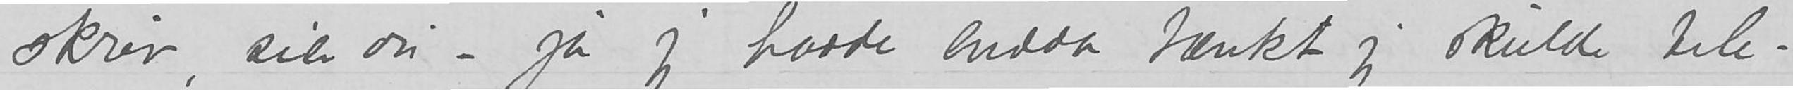skriv, sier du - ja jeg hadde endda tænkt jeg skulde tele-
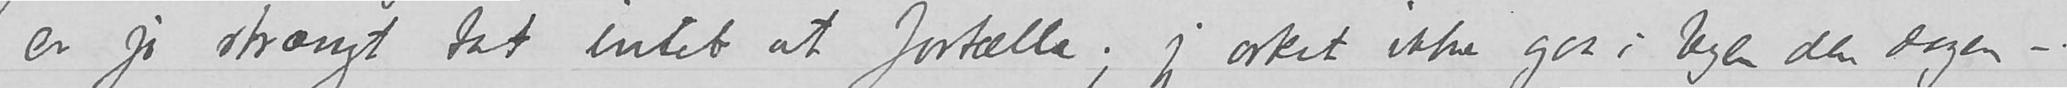er jo strængt tat intet at fortælle; jeg orket ikke gaa i byen den dagen -.
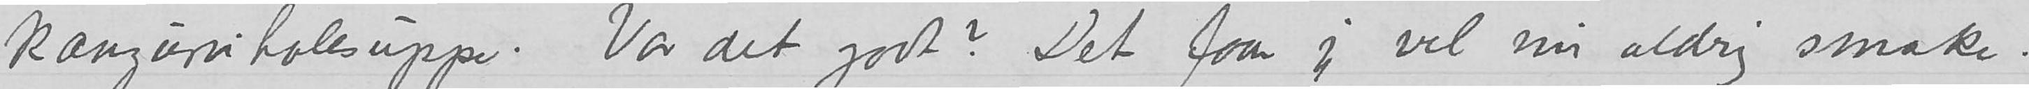kænguruhalesuppe. Var det godt? Det faar jeg vel nu aldrig smake.
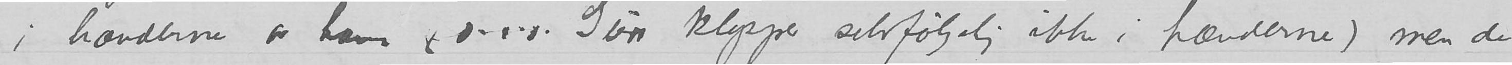i hænderne av ham. (d.v.s. Guro klapper selvfølgelig ikke i hænderne), men de
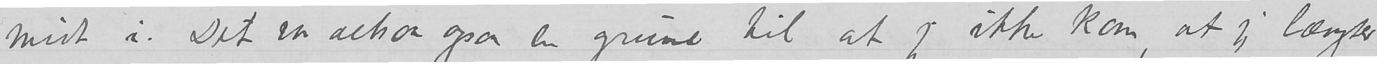midt i. Det er altsaa ogsaa en grund til at jeg ikke kom, at jeg længter
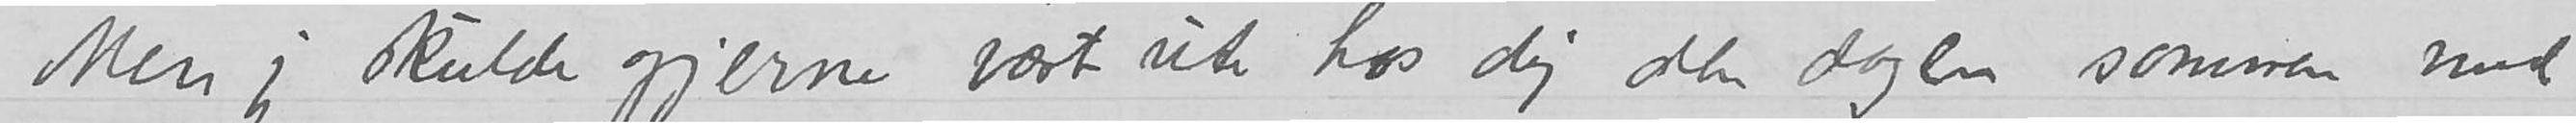Men jeg skulde gjerne vært ute hos dig den dagen sammen med
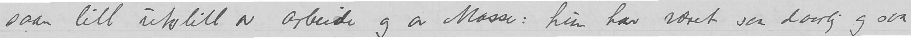saan litt utslitt av arbeide og av Mosse: hun har været saa daarlig og saa
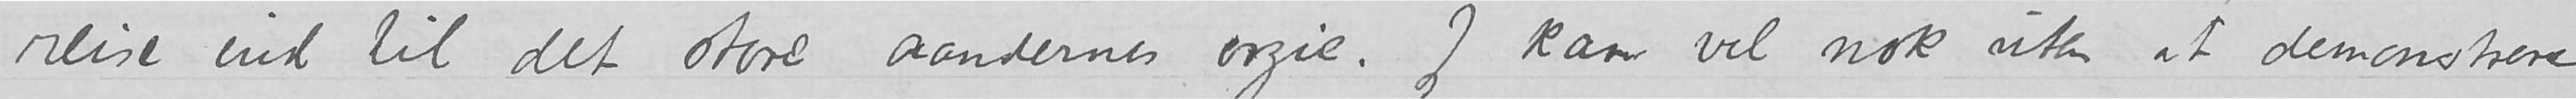reise ind til det store aandernes orgie. Jeg kan vel nok uten at demonstrere
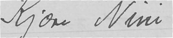Kjære Nini,
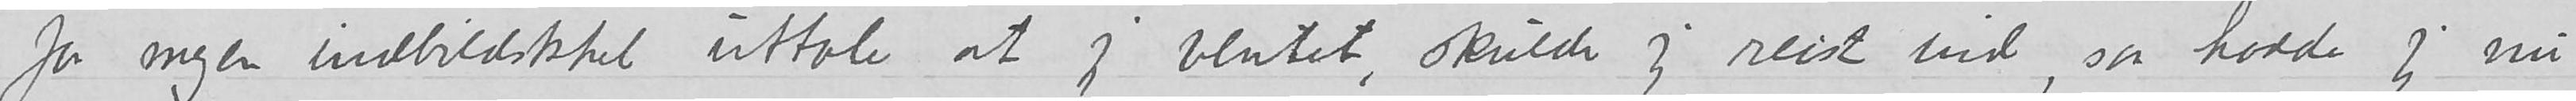for megen indbildskhet uttale at jeg ventet, skulde jeg reist ind, saa hadde jeg nu
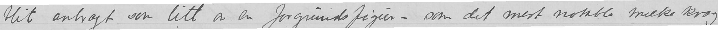blit anbragt som litt av en forgrundsfigur - som det mest notable mælke kvæg
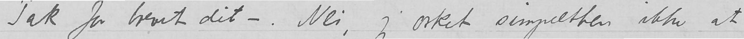Tak for brevet dit -. Nei, jeg orket simpelthen ikke at
